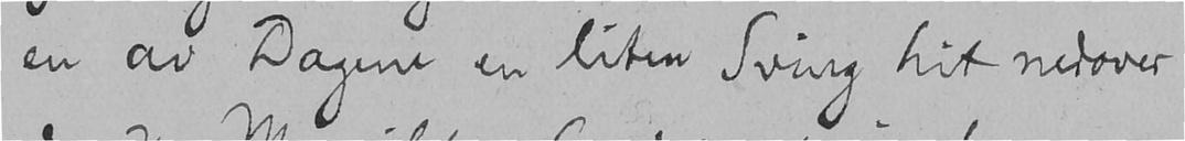en av Dagene en liten Sving hit nedover
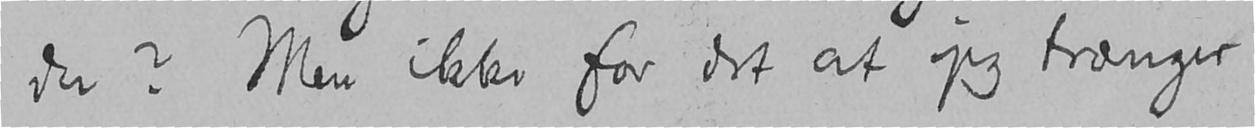du? Men ikke for det at jeg trænger
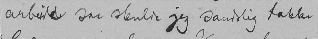arbeide saa skulde jeg sandelig takke
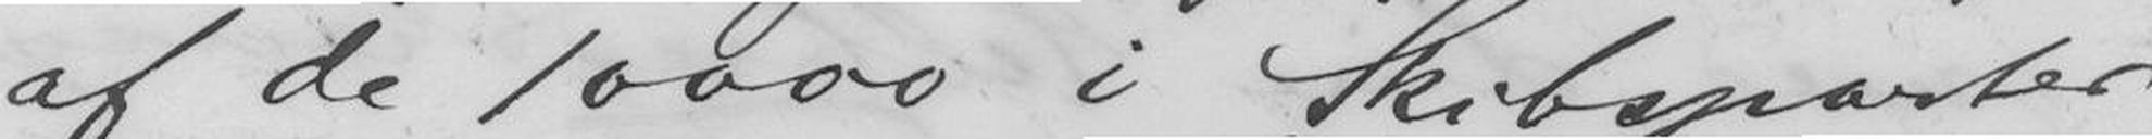af de 10000 i Skibsparter
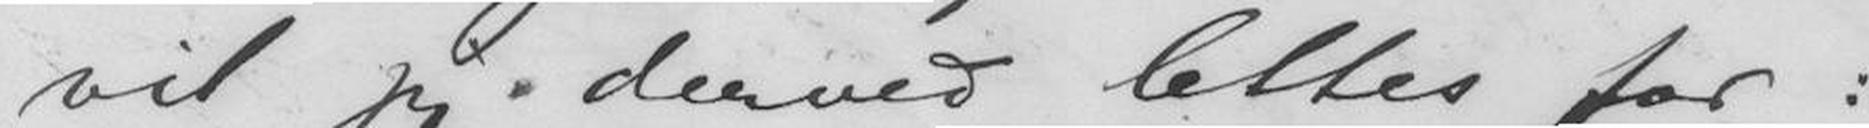vil jeg derved lettes for:
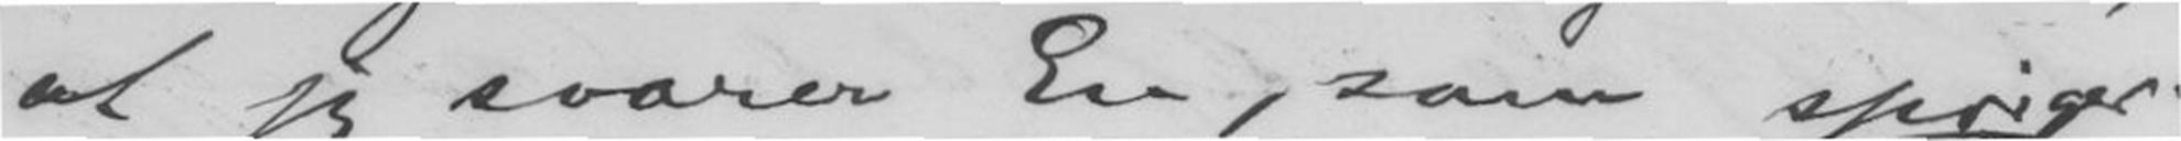at jeg svarer En, som spørger.
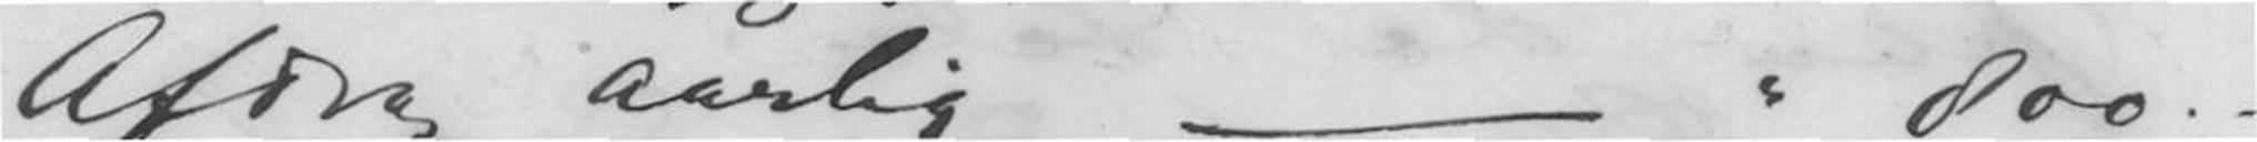Afdrag Aarlig____________" 800.-
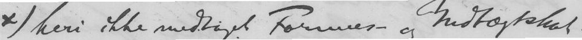x) heri ikke medtaget Formues- og Indtægtskat.
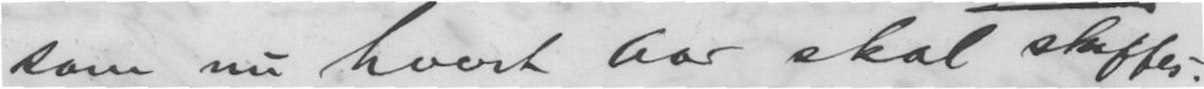som nu hvert Aar skal skaffes.
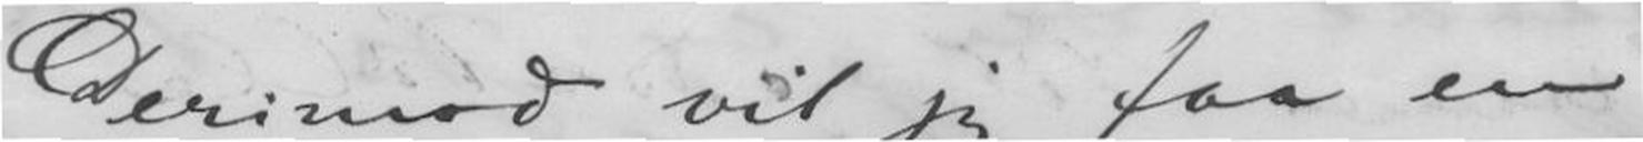Derimod vil jeg faa en
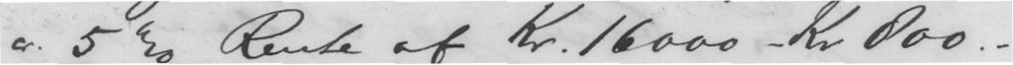ca. 5% Rente af Kr. 16000Kr 800.-
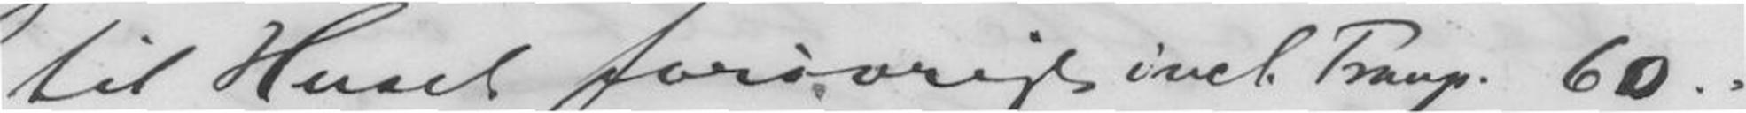til Huset forøvrigt incl.Transp. 60.-
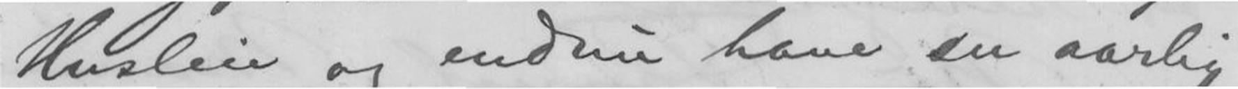Husleie og endnu have en aarlig
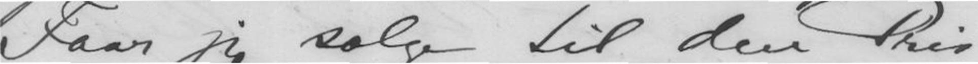Faar jeg sælge til den Pris
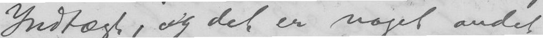Indtægt, sig det er noget andet
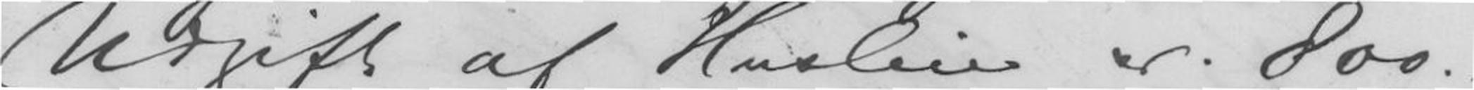Udgift af Husleie ca. 800.-
